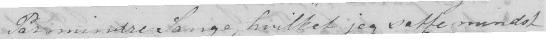Par mindre Sange hvilket jeg satte mindst
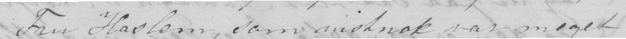Fru Haslem, som vistnok var meget
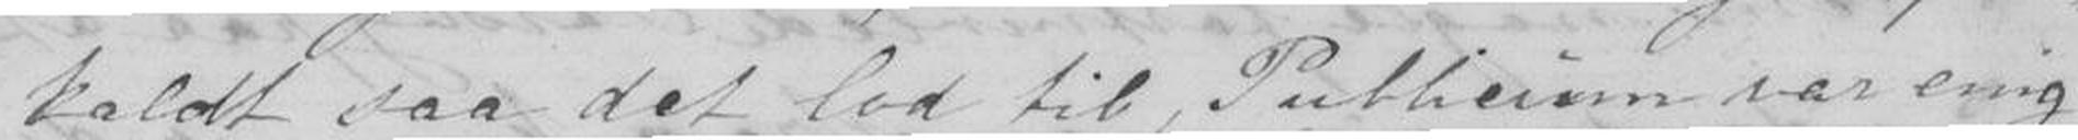kaldt saa det lod til, publicum var enig
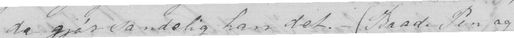da gjør sandelig han det. -
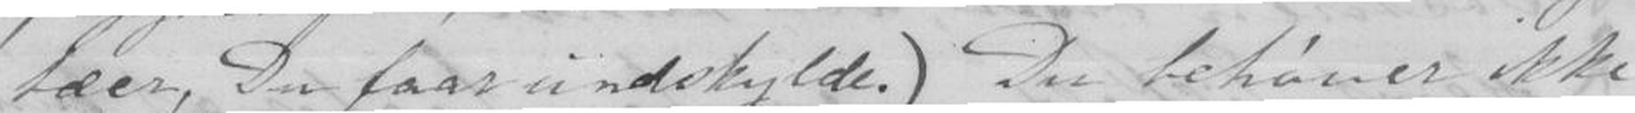faer, Der faar vi undskylde. Du behøver ikke
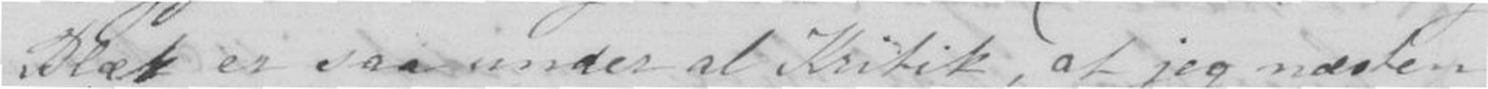er saa under at Kritk, at jeg næsten
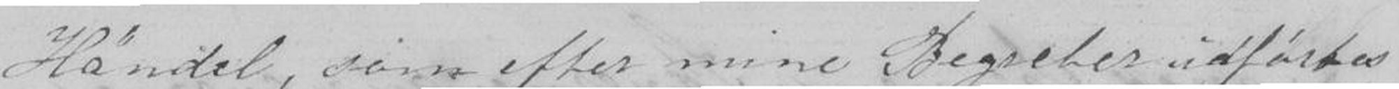Händel, som efter mine Begreber udføres
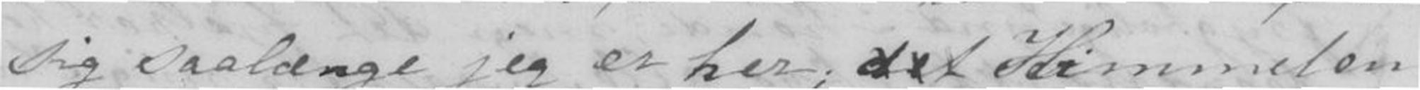sig saalænge jeg er her; det Himmelen
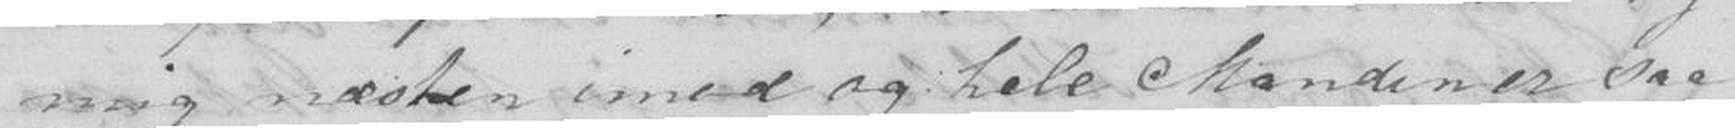mig næsten imod og hele Manden er saa
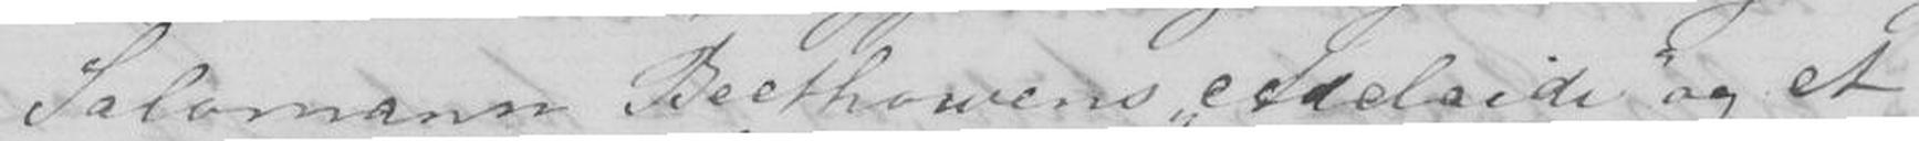Salomann, Beethowens Adeleide og et
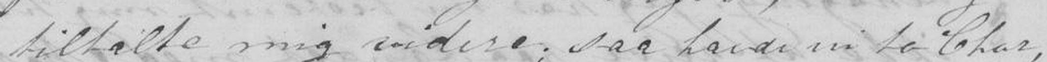tiltalte mig videre; saa havde vi to Chor,
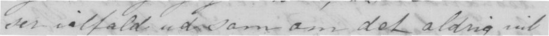ser ialfald ud som om det aldrig vil
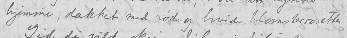hjemme, dækket med røde og hvide blomsterrosetter!
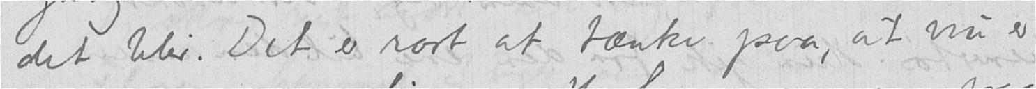det blir. Det er rart at tænke paa, at nu er
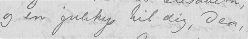og en julekys til dig, Dea,
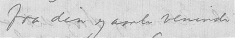fra din gamle veninde
